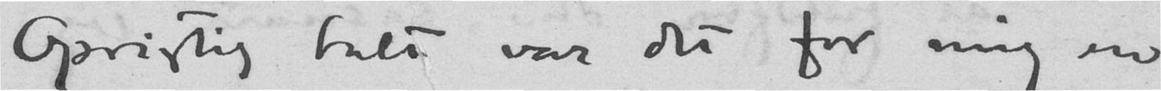Oprigtig talt var det for mig en
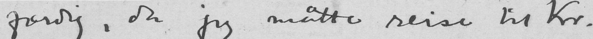færdig, da jeg måtte reise til Kr.
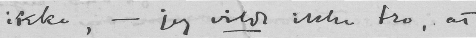ikke, - jeg vilde ikke tro, at
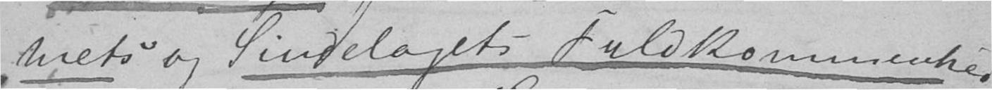mets og Sindelagets Fuldkommenhed.
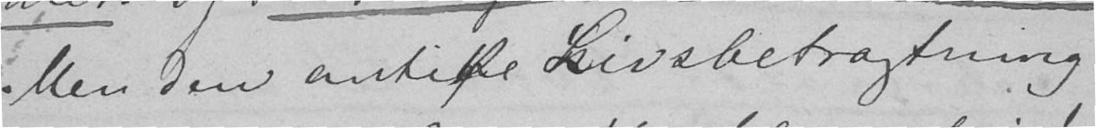Men den antike Livsbetragtning,
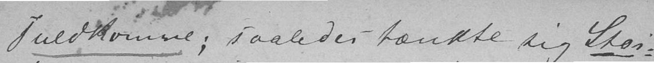fuldkomne; saaledes tænkte sig Stoi-
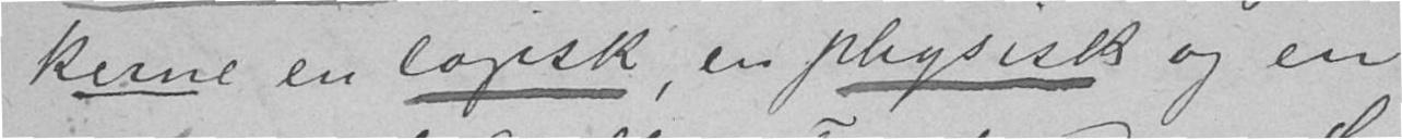kerne en logisk, en physisk og en
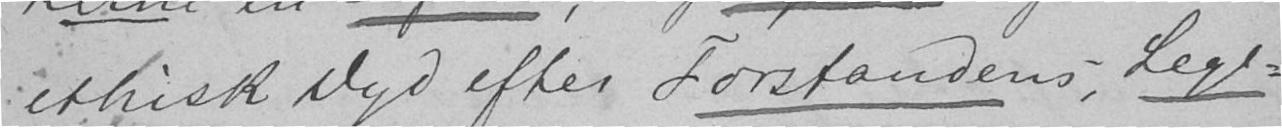ethisk Dyd efter Forstandens, Lege-
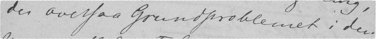der oversaa Grundproblemet i den
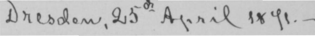Dresden, 25de April 1871.-
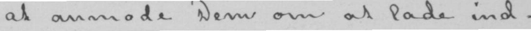at anmode Dem om at lade ind-
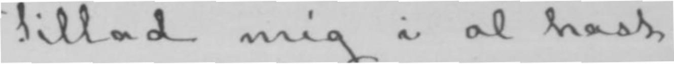Tillad mig i al hast
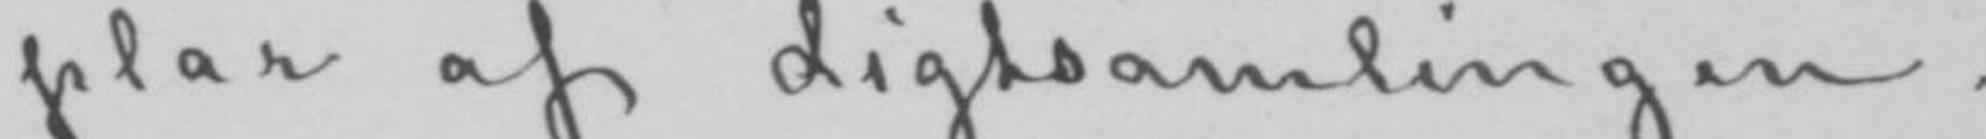plar af digtsamlingen.
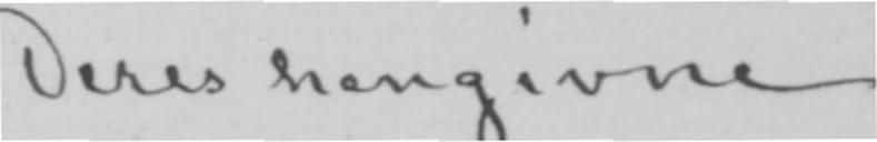Deres hengivne
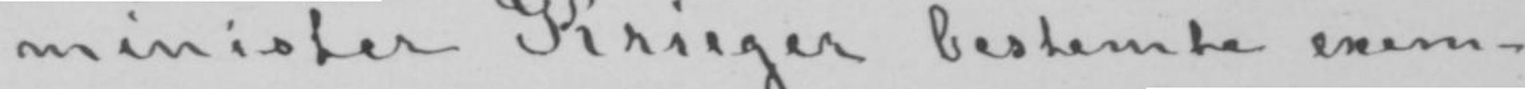minister Krieger bestemte exem-
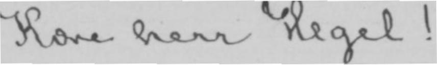Kære herr Hegel!
